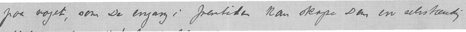paa noget, som De engang i fremtiden kan skape som en selvstændig
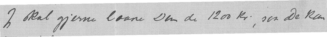Jeg skal gjerne laane Dem de 1200 kr., saa De kan
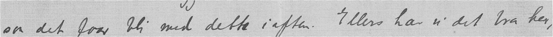saa det faar bli med dette i aften. Ellers har vi det bra her,
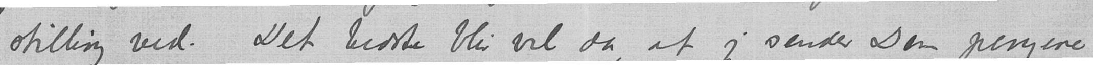stilling ved. Det bedste blir vel da, at jeg sender Dem pengene
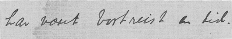har været bortreist en tid.
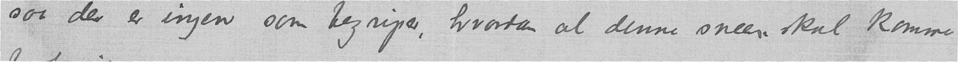saa der er ingen som begriper, hvordan al denne sneen skal komme
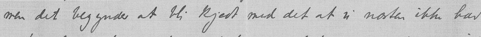men det begynder at bli kjedt med det at vi næsten ikke har
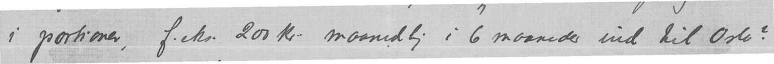i portioner, f.eks. 200 kr. maanedlig i 6 maaneder ind til Oslo?
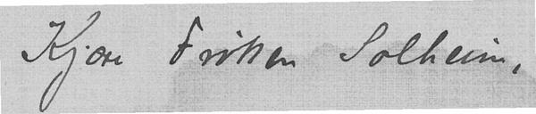Kjære Frøken Solheim,
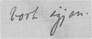bort igjen.
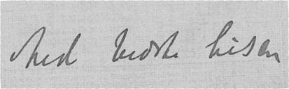Med bedste hilsen
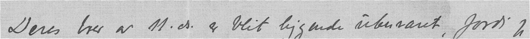Deres brev av 11.ds. er blit liggende ubesvaret, fordi jeg
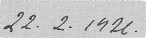2.2.1926.
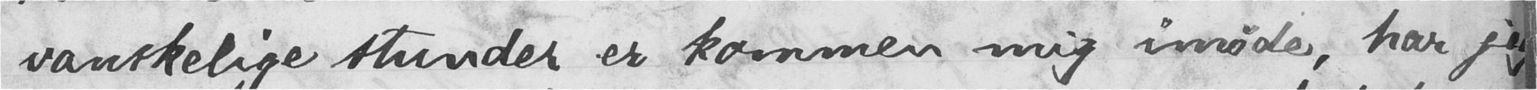vanskelige stunder er kommen mig imøde, har jeg,
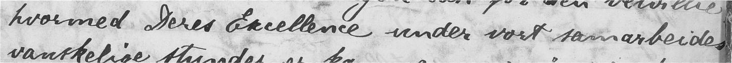hvormed Deres Excellence under vort samarbeides
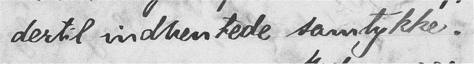dertil indhentede samtykke.
